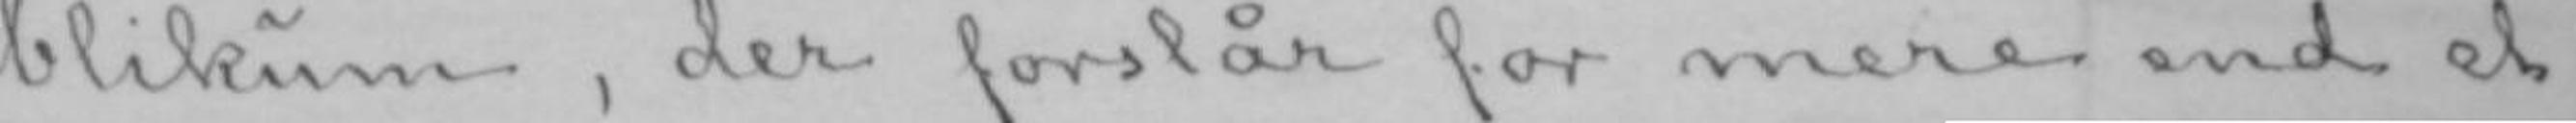blikum, der forslår for mere end et
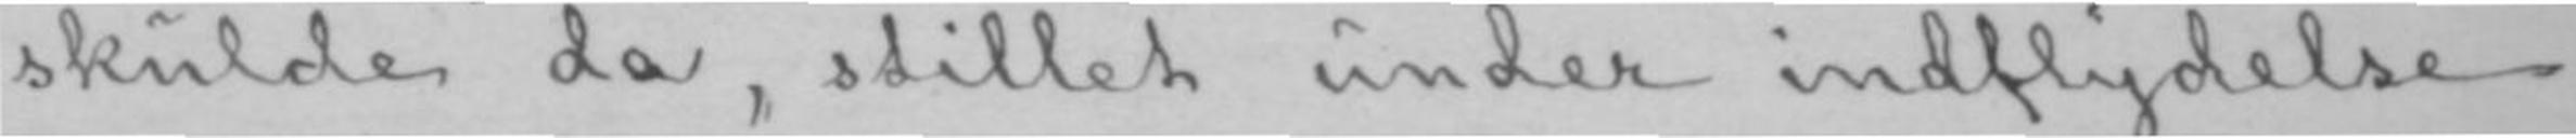skulde da, stillet under indflydelse
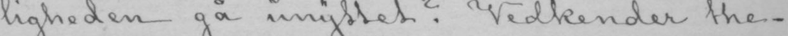ligheden gå unyttet? Vedkender the-
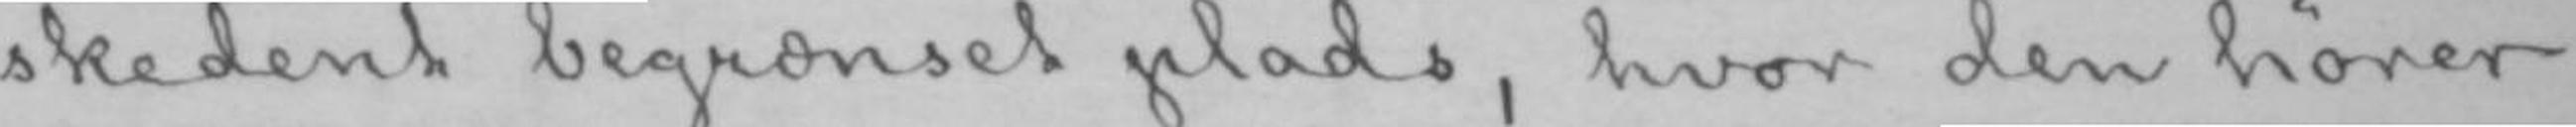skedent begrænset plads, hvor den hører
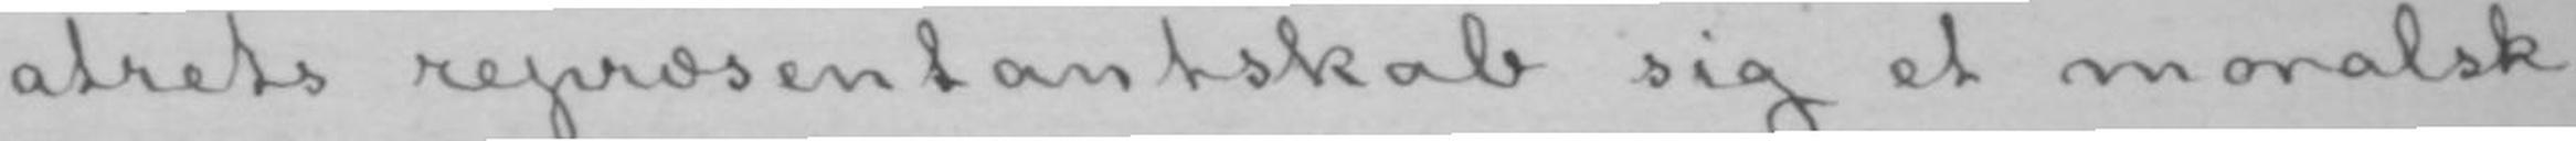atrets repræsentantskab sig et moralsk
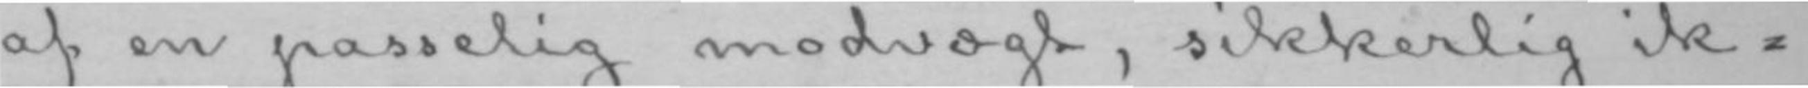af en passelig modvægt, sikkerlig ik-
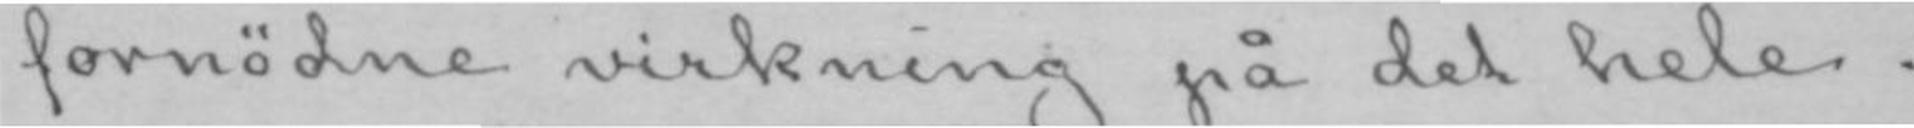fornødne virkning på det hele.
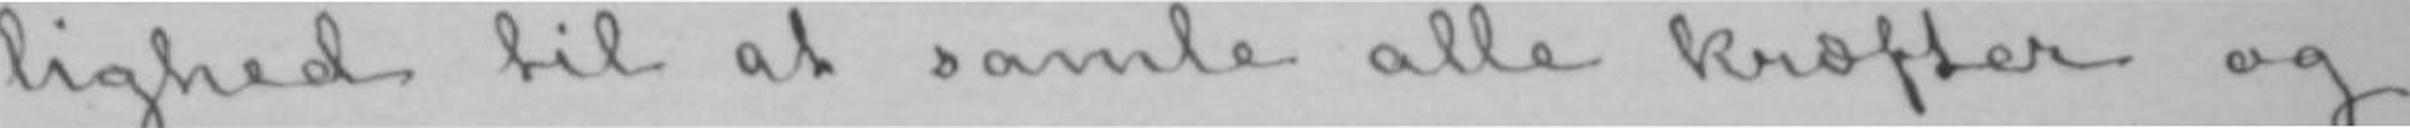lighed til at samle alle kræfter og
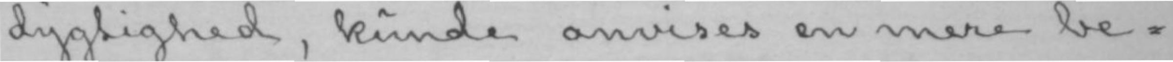dygtighed, kunde anvises en mere be-
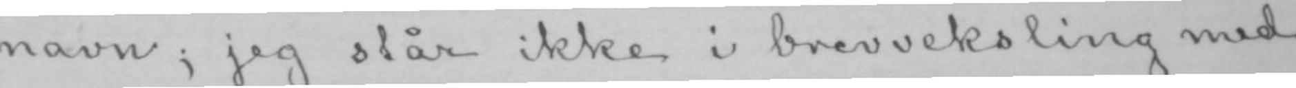navn; jeg står ikke i brevveksling med
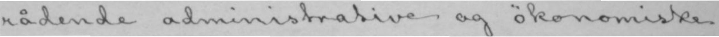rådende administrative og økonomiske
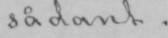sådant.
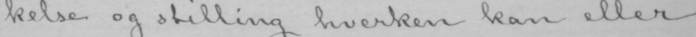kelse og stilling hverken kan eller
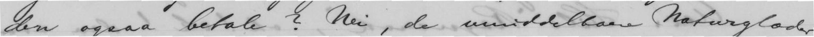den ogsaa betale? Nei, de umiddelbare Naturglæder,
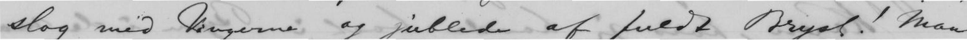slog med Vingerne og jublede af fuldt Bryst! Maa
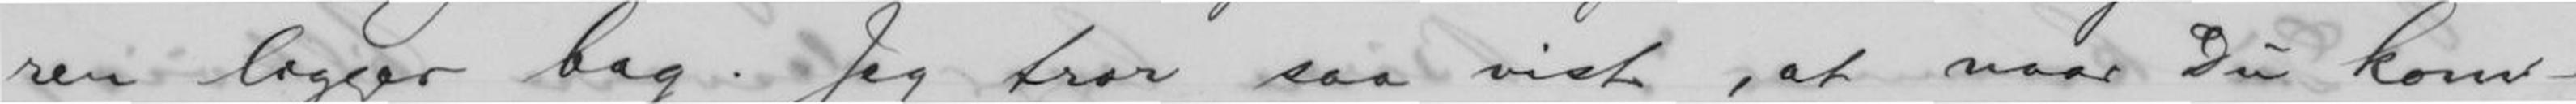ren ligger bag. Jeg tror saa vist, at naar Du kom-
